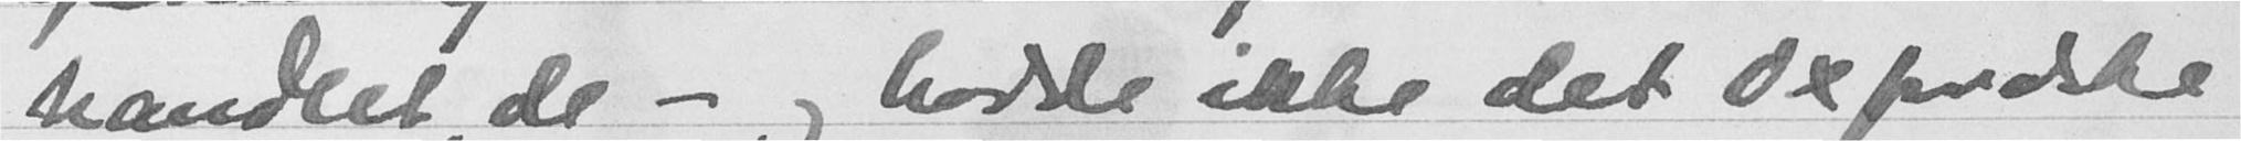handlet, de - og hadde ikke det Oxfordske
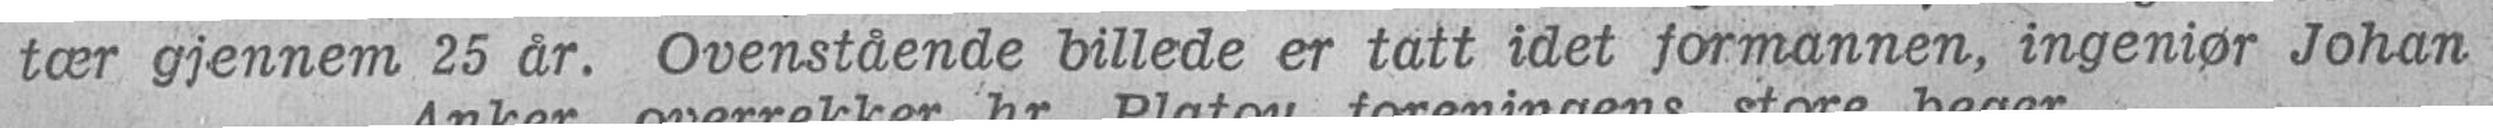tær gjennem 25 år. Overstående billede er tatt idet formannen, ingeniør Johan
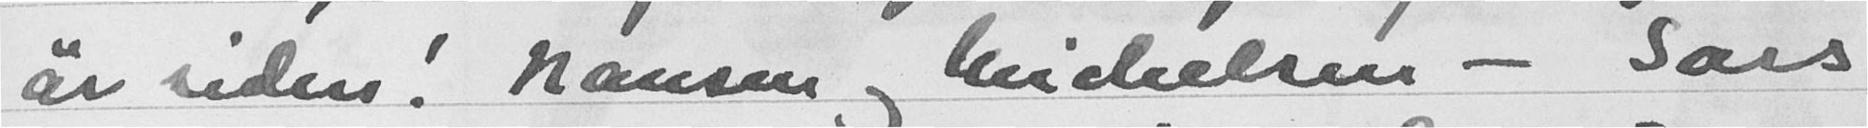år siden. Nansen og Michelsen - Sars
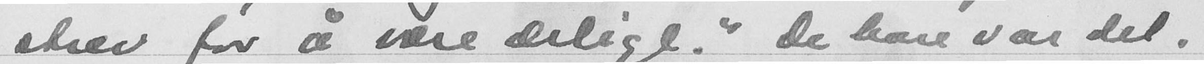strev for å være "ærlige." De bare var det.
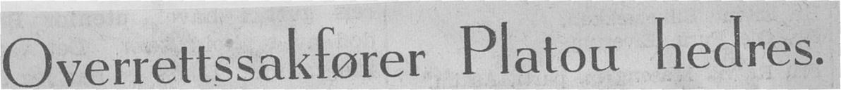Overrettssakfører Platou hedres.
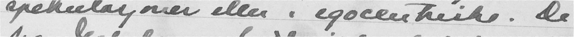spekulasjoner eller i egocentriske. De
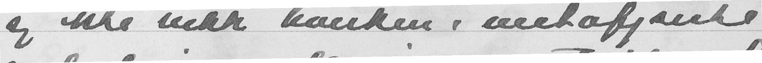sig ikke vekk hverken i metafysiske
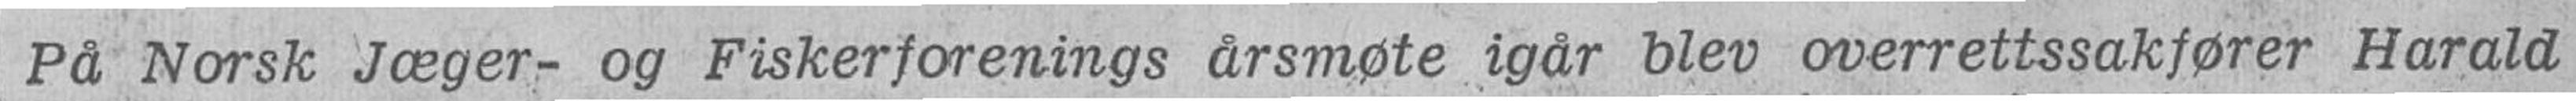På norsk Jæger- og Fiskerforenings årsmøte igår blev overrettssakfører Harald
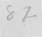87
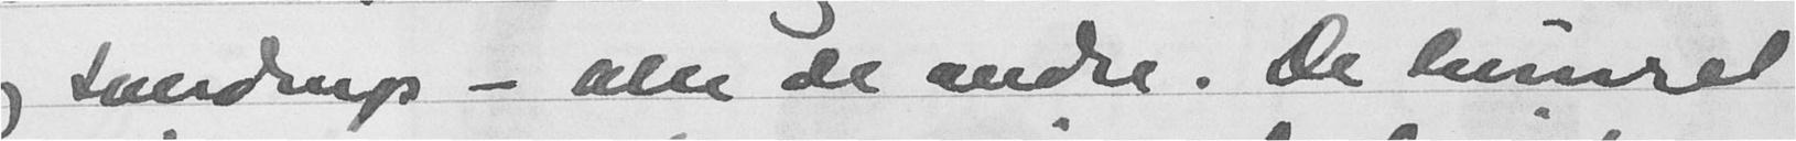og Sverdrups - alle de andre. De limret
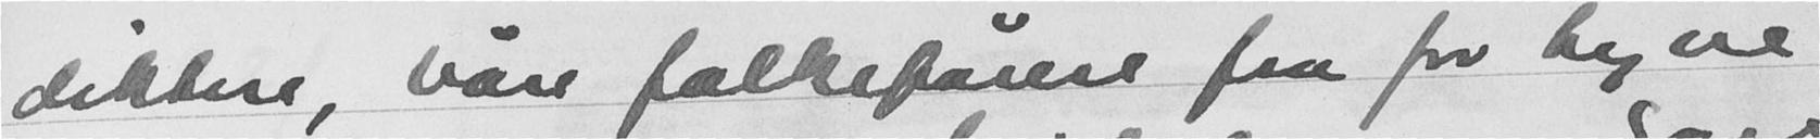diktere, våre folkeførere fra for tyve
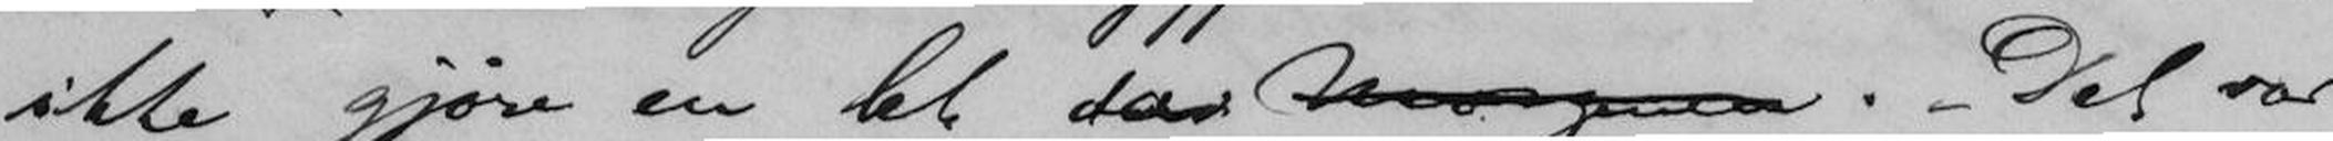ikke gjøre en let da Morgen. - Det var
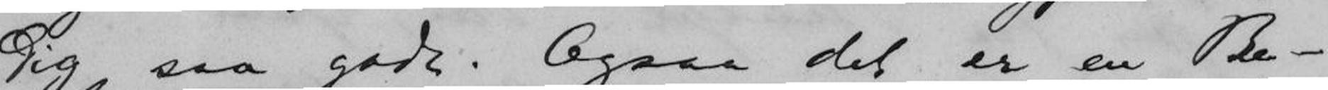Dig saa godt. Ogsaa det er en Be-
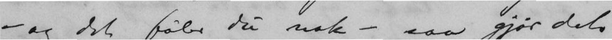- og det føler du nok - saa gjør det
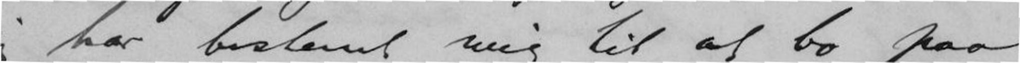jeg har bestemt mig til at bo paa
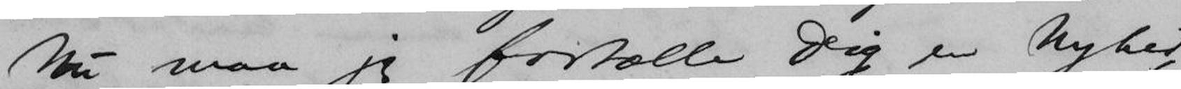Nu maa jeg fortælle Dig en Nyhed,
